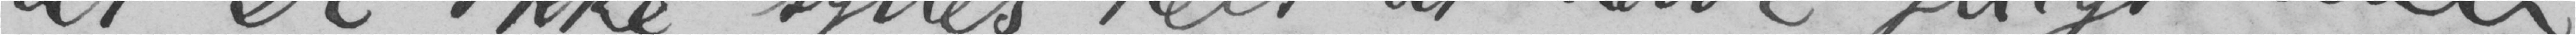at De ikke synes helt at have fulgt min
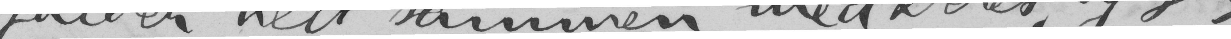falder helt sammen med Deres, og jeg
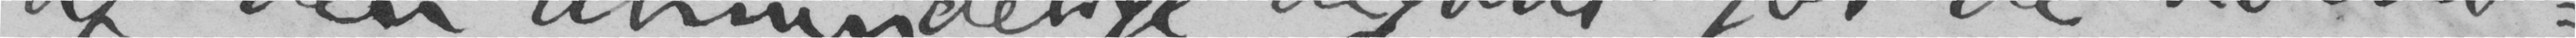af den almindelige dégoût for de homo-
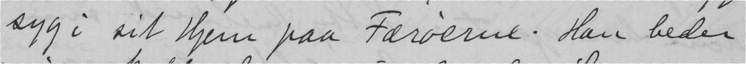syg i sit Hjem paa Færøerne. Han beder
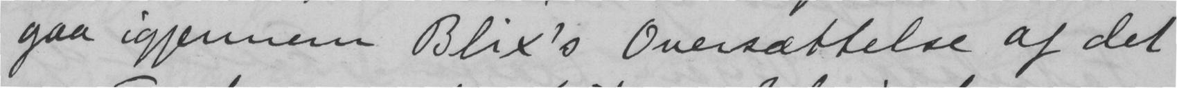gaa igjennem Blix's Oversættelse af det
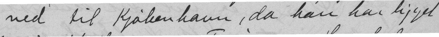ned til Kjøbenhavn, da han har ligget
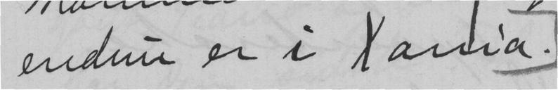endnu er i Xania.
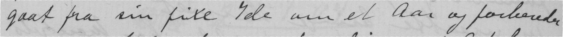gaat fra sin fixe Ide om et Aar og forbereder
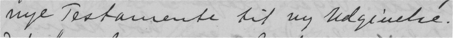nye Testamente til ny Udgivelse.
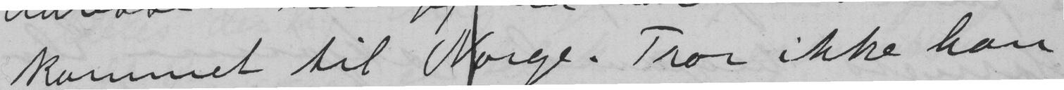kommet til Norge. Tror ikke han
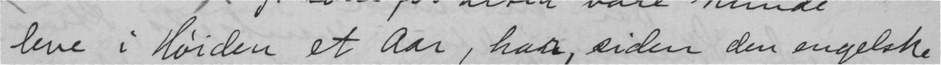leve i Høiden et Aar, har, siden den engelske
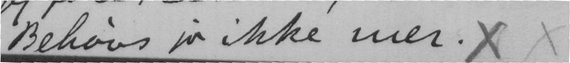Behøvs jo ikke mer. xx
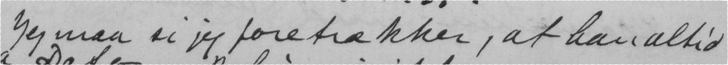Jeg maa si jeg foretrækker, at han altid
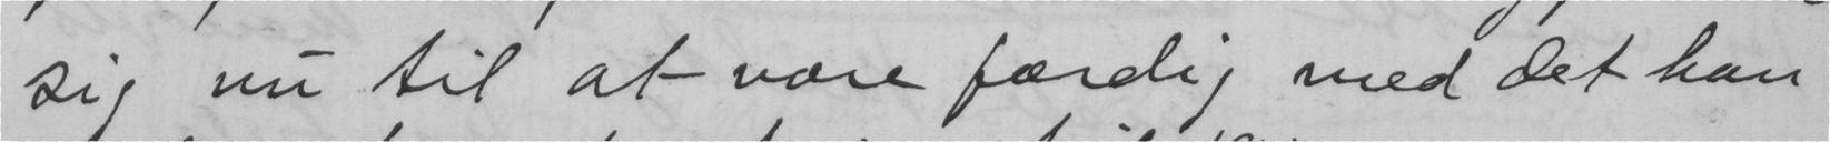sig nu til at være færdig med det han
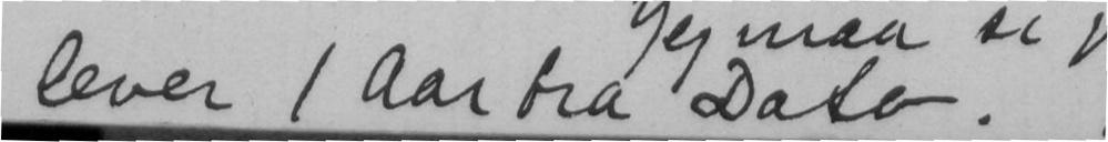lever / Aar bra Dato.
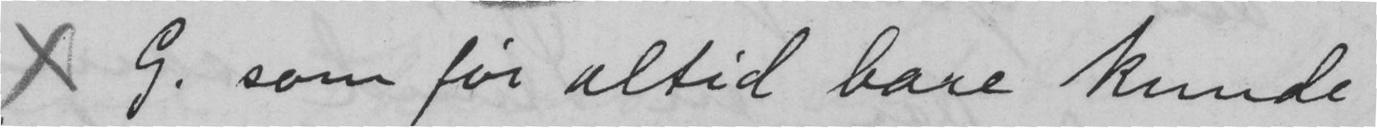x G. som før altid bare kunde
